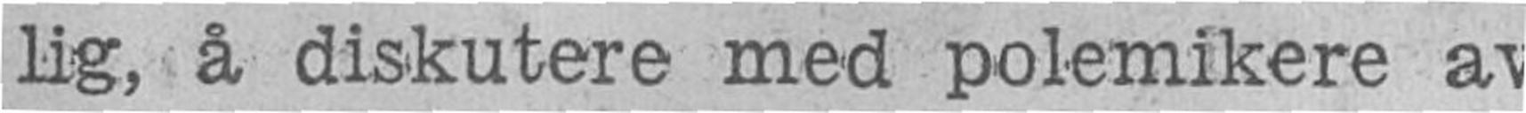lig, å diskutere med polemikere av
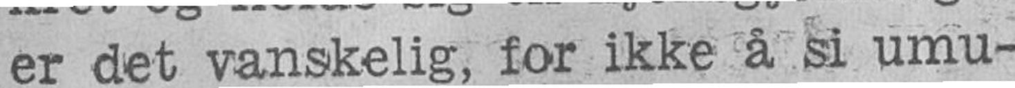er det vanskelig, for ikke å si umu-
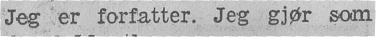Jeg er forfatter. Jeg gjør som
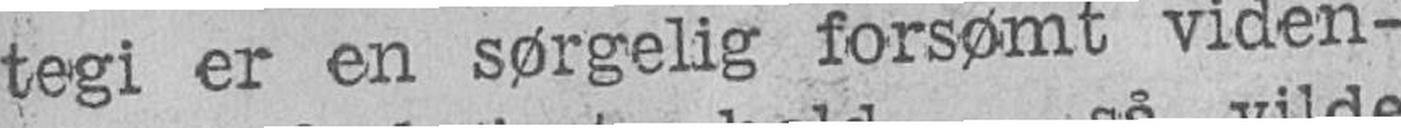tegi er en sørgelig forsømt viden-
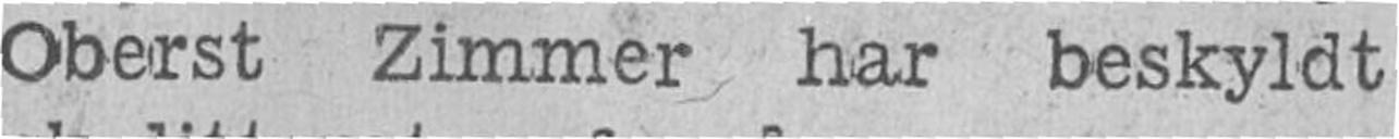Oberst Zimmer har beskyldt
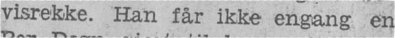visrekke. Han får ikke engang en
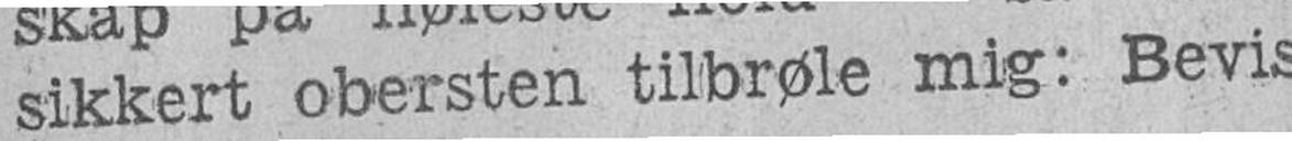sikkert obersten tilbrøle mig: Bevis
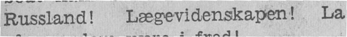Russland! Lægevidenskapen! La
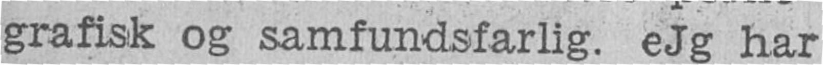grafisk og samfundsfarlig. eJg har
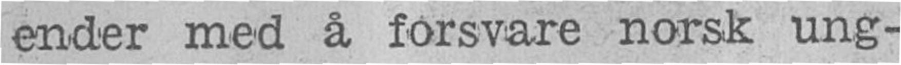ender med å forsvare norsk ung-
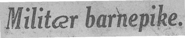Militær barnepike.
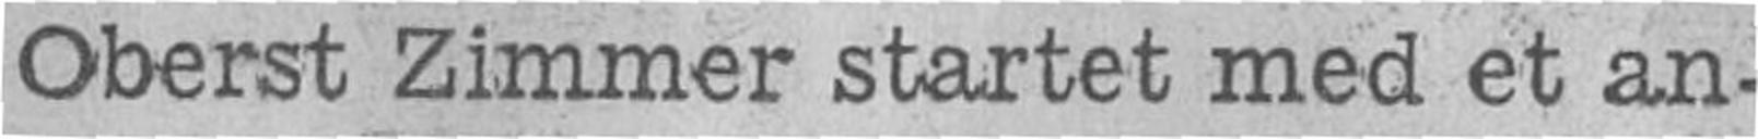Oberst Zimmer startet med et an-
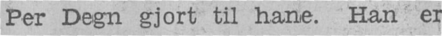Per Degn gjort til hane. Han er
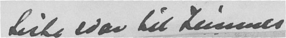Siste svar til Zimmer
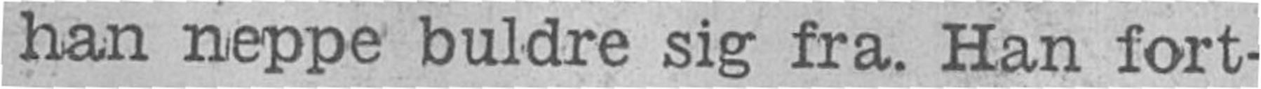han neppe buldre sig fra. Han fort-
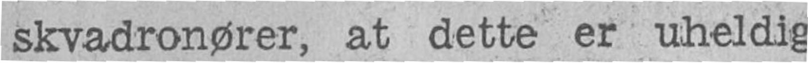skvadronører, at dette er uheldig
